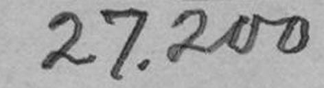27.200
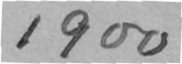1900
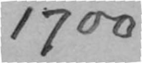1700
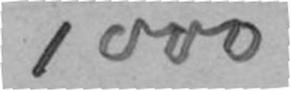1000
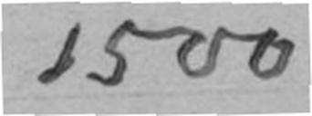1500
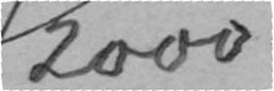2000
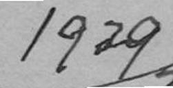1929
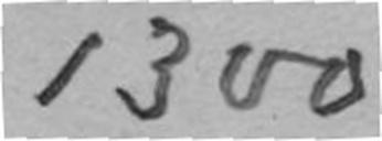1300
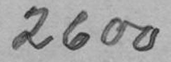2600
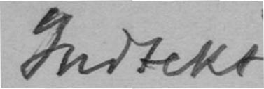Indtekt
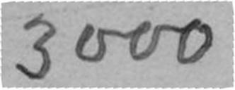3000
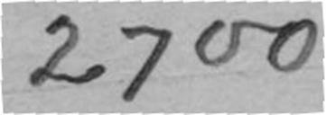2700
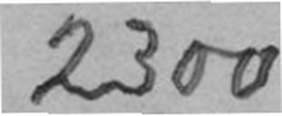2300
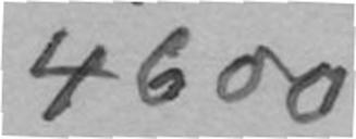4600
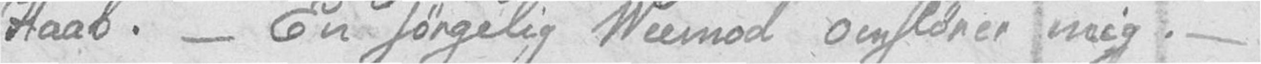Haab. – En sørgelig Veemod omslører mig. –
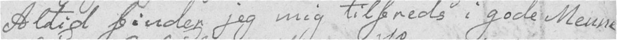Altid finder jeg mig tilfreds i gode Menne
